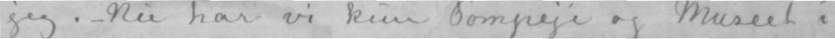jeg.- Nu har vi kun Pompeji og Museet i
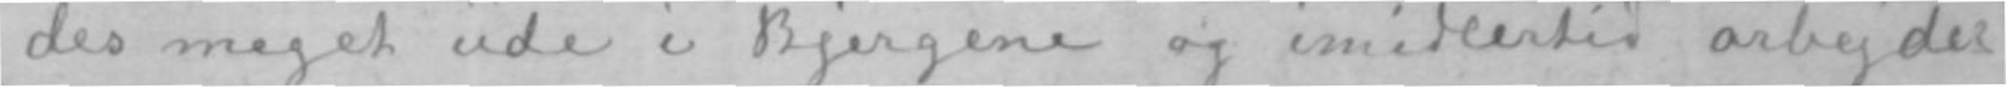des meget ude i Bjergene og imidlertid arbejder
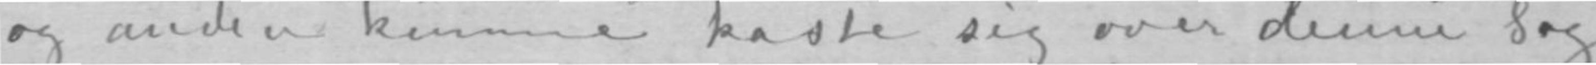og anden kunne kaste sig over denne Sag
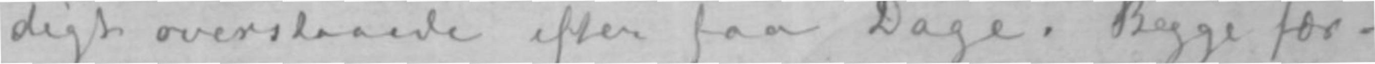digt overstaaede efter faa Dage. Begge fær-
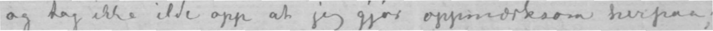og tag ikke ilde opp at jeg gjør oppmærksom herpaa;
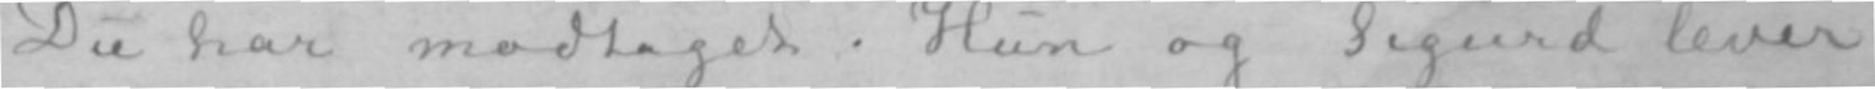Du har modtaget. Hun og Sigurd lever
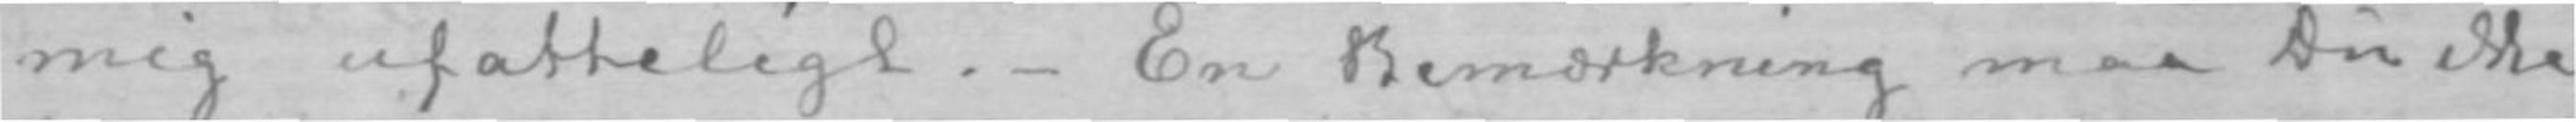mig ufatteligt.- En Bemærkning maa Du ikke
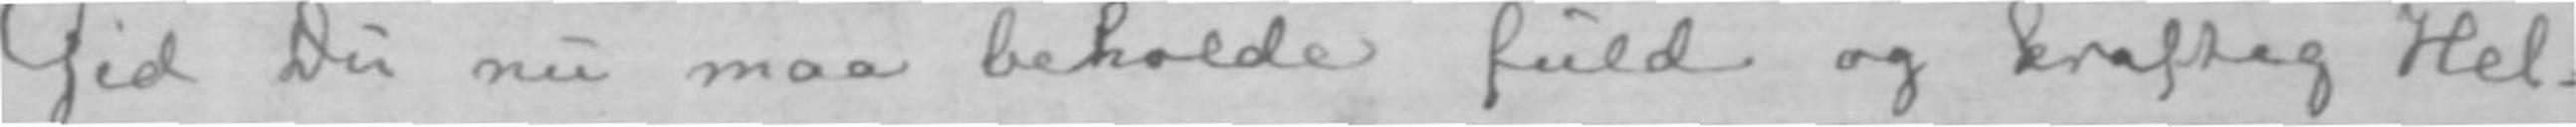Gid Du nu maa beholde fuld og kraftig Hel-
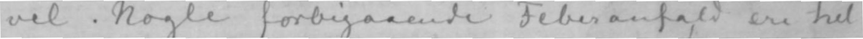vel. Nogle forbigaaende Feberanfald ere hel-
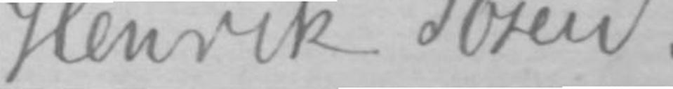Henrik Ibsen.
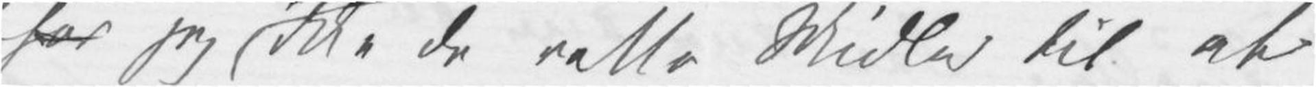har jeg ikke de rette Midler til at
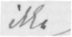ikke
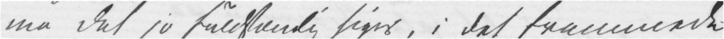må det jo fuldstændig siges, i det fremmede
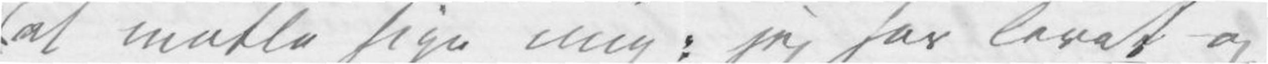at måtte sige mig: jeg har levet og
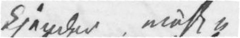kjender måske
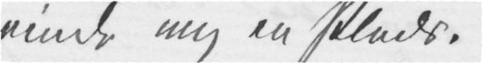vinde mig en Plads.
